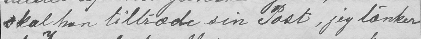skal han tiltræde sin Post, jeg tænker
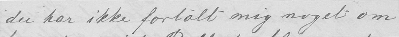du har ikke fortalt mig noget om
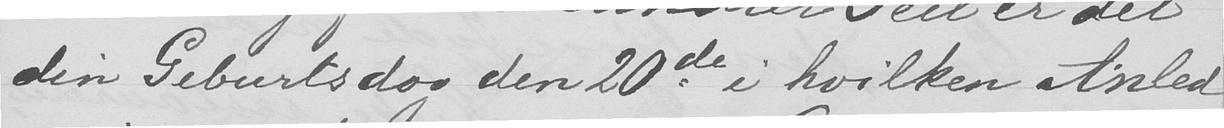din Geburtsdag den 20de i hvilken Anled-
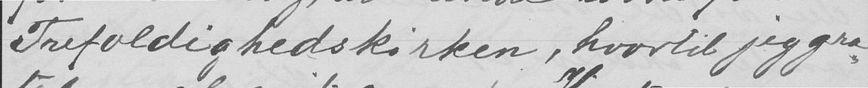Trefoldighedskirken, hvortil jeg gra-
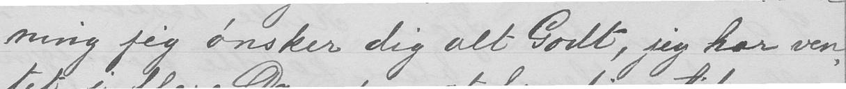ning jeg ønsker dig alt Godt, jeg har ven-
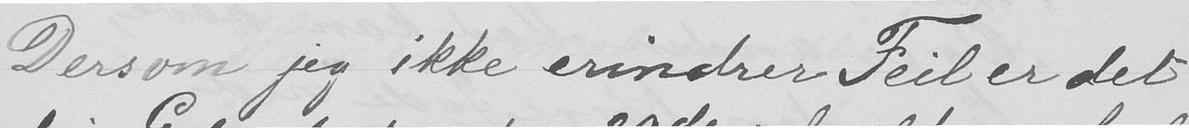Dersom jeg ikke erindrer Feil er det
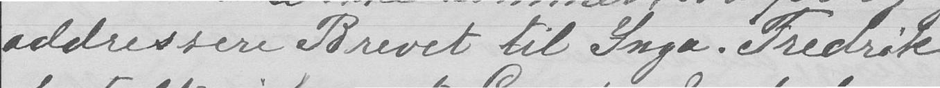addressere Brevet til Inga. Fredrik
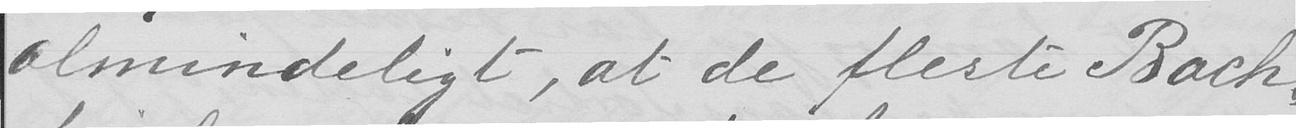almindeligt, at de fleste Bach-
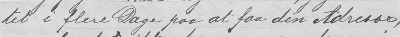tet i flere Dage paa at faa din Adresse,
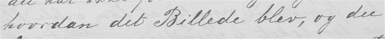hvordan det Billede blev, og du
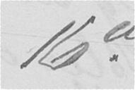16de
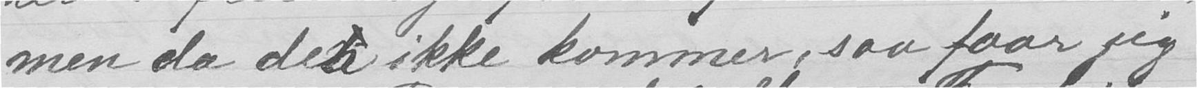men da det ikke kommer, saa faar jeg
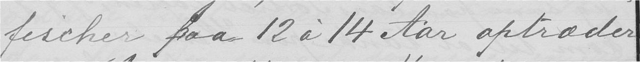fischer paa 12 à 14 Aar optræder
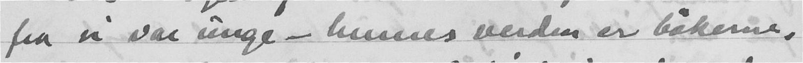fra vi var unge - hennes verden er bøkerne,
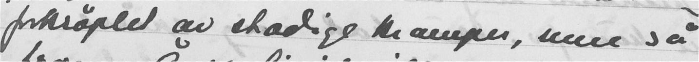forkrøplet av stadige kramper, men så
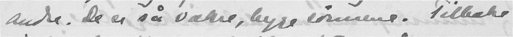andre. De er så vakre, begge sønnene. Tilbake
